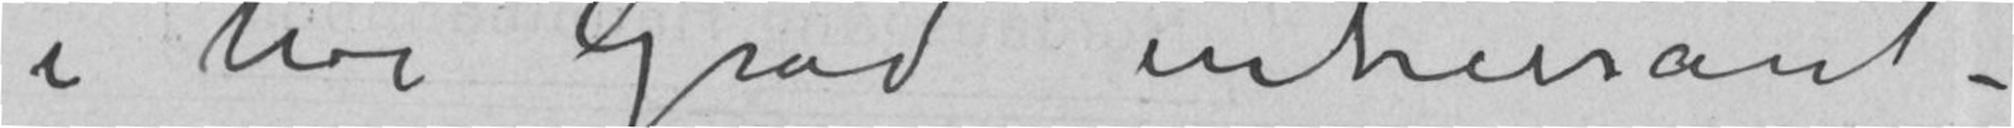i høi Grad interessant –
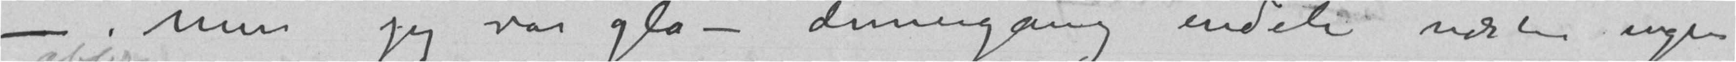– Men jeg var gla – dennegang endeli næsten ingen
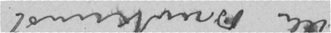eller Brevkunst
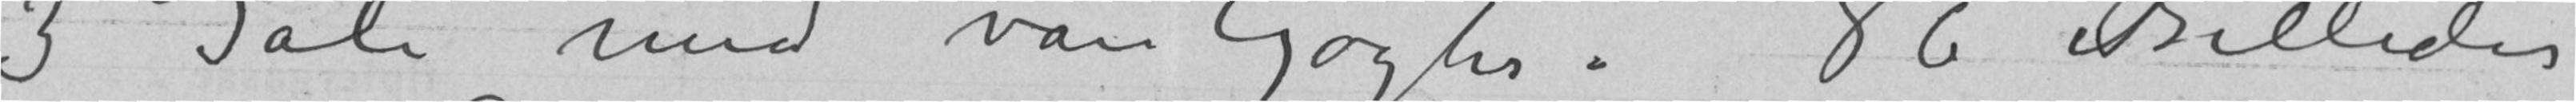3 Sale med Van Goghs! 86 Billeder
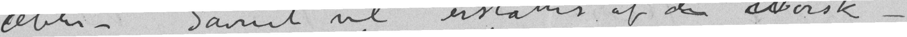Æbler – Savnet vil erstattes af de Norske –
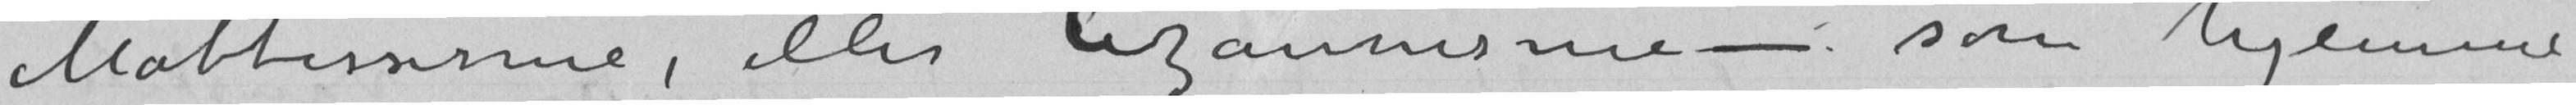Mattissisme, eller Cezanneisme – som hjemme
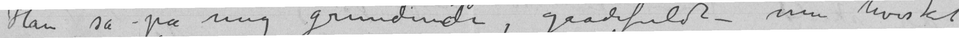Han så på mig grundende, gaadefuldt, men hvisket
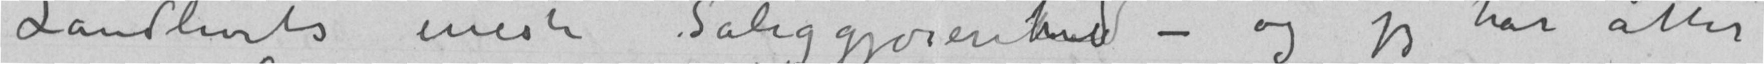Landlivets eneste Saliggørenhed – og jeg har atter
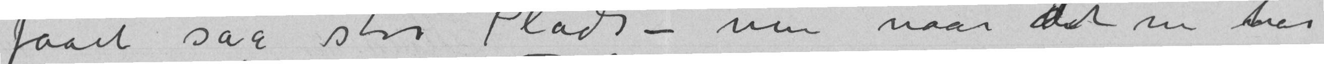faaet så stor Plads – men naar det nu har
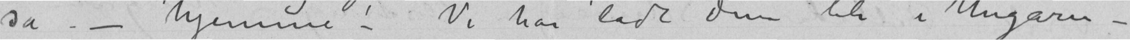så – hjemme – Vi har ladt dem bli i Ungarn –
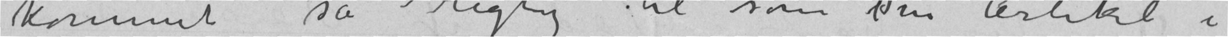kommet så rigtig til som Din Artikel i
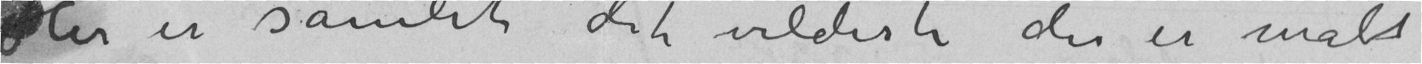Her er samlet det vildeste der er malt
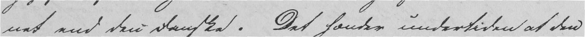net end den danske. Det hænder undertiden at den
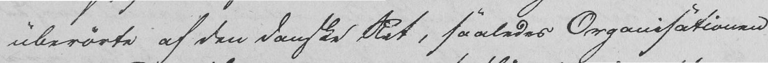uberørte af den danske Ret, saaledes Organisationen
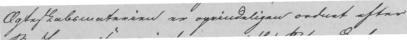Ægteskabsmaterien er oprindeligen ordnet efter
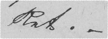Ret. -
